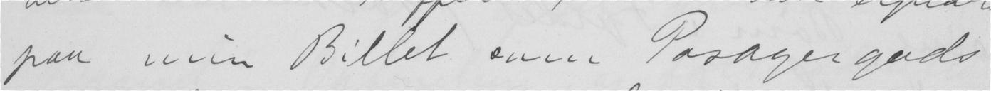paa min Billet som Pasager gods
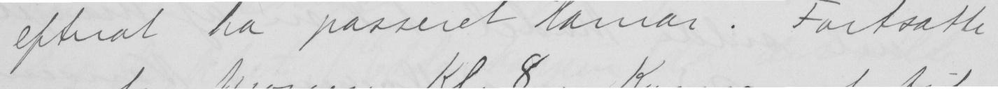efterat ha passeret Hamar. Fortsatte
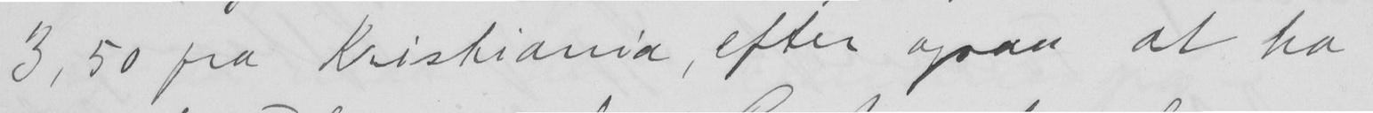3,50 fra Kristiania, efter ogsaa at ha
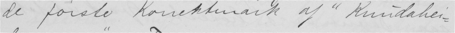de første Korekturark af "Knudahei-
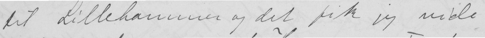til Lillehammer og det fik jeg vide
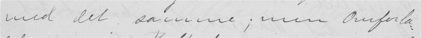med det samme; men Omforlag
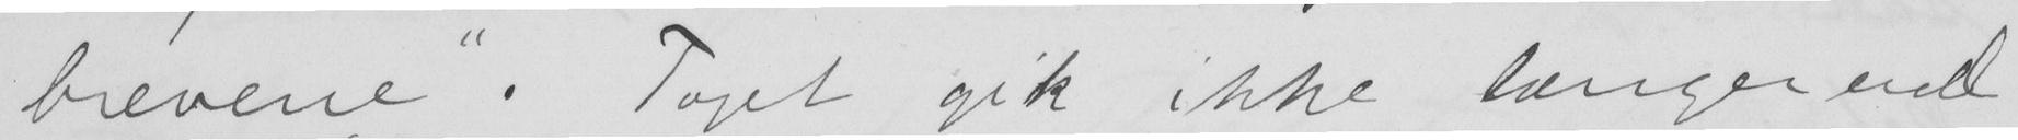brevene". Toget gik ikke længer end
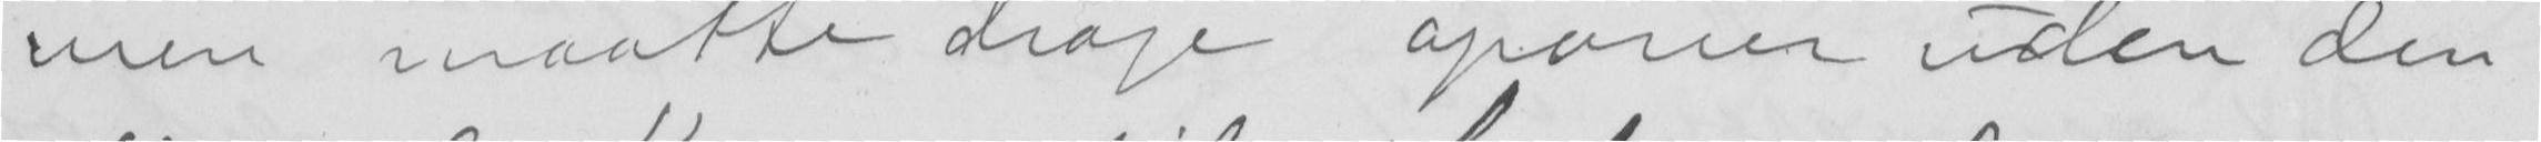men maatte drage opover uden den
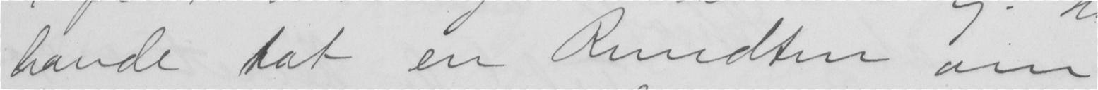havde hat en Rundten om
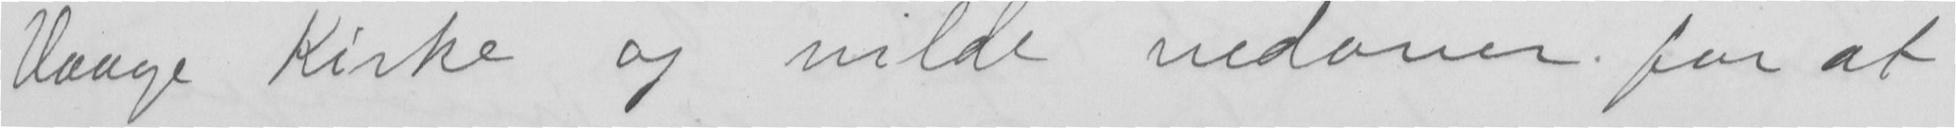Vaage Kirke og vilde nedover for at
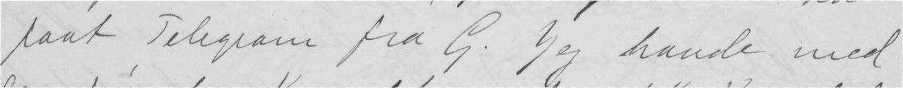faat Telegrom fra G. Jeg havde med
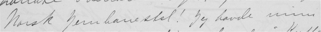Norsk Jembanestel! Jeg havde min
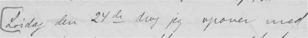Lørdag den 24de drog jeg opover med
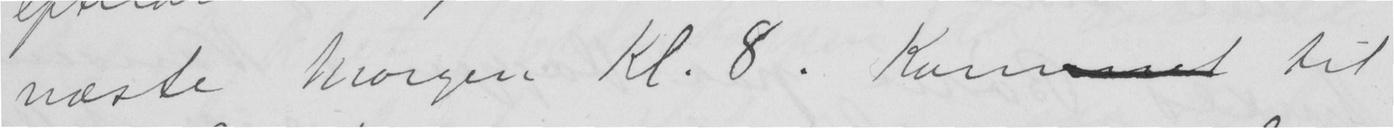næste Morgen Kl. 8. Kommet til
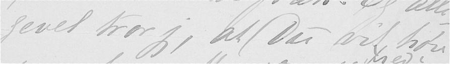gevel tror jeg, at Du vil, høre
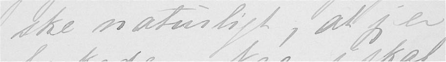ske naturligt, at jeg er
